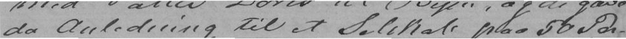da Anledning til et Selskab paa 50 Per-
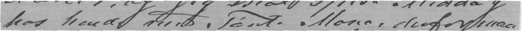hos hende med Tante Mone, derfor maa
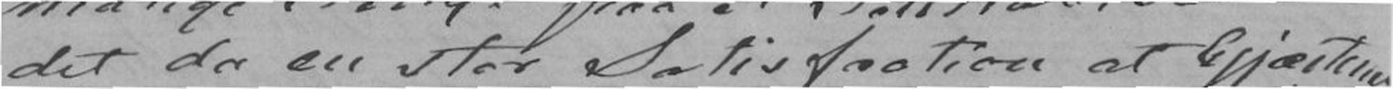det da en stor Satisfaction at Gjæstene
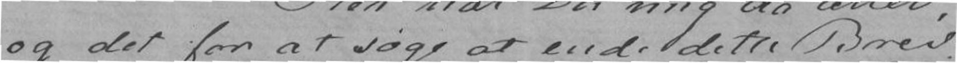og det for at søge at ende dette Brev
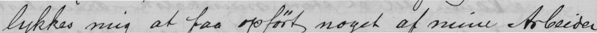lykkes mig at faa opført noget af mine Arbeider
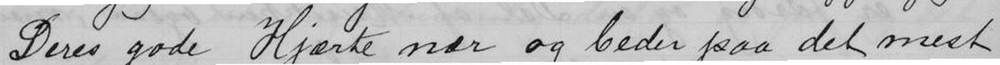Deres gode Hjærte nær og beder paa det mest
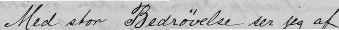Med stor Bedrøvelse ser jeg af
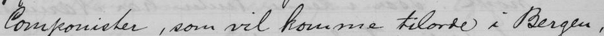Componister, som vil komme tilordet i Bergen,
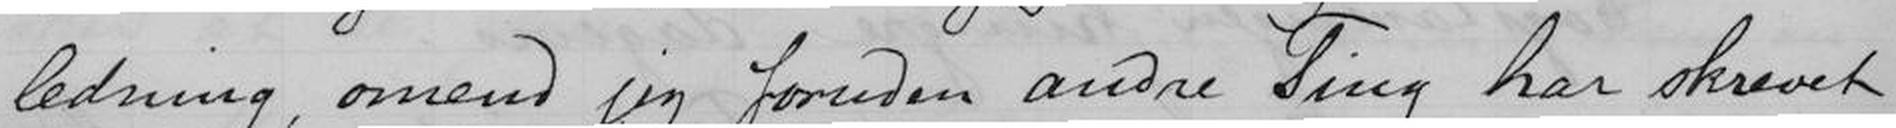ledning, omend jeg foruden andre Ting har skrevet
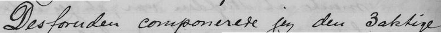Desforuden componerede jeg den 3aktige
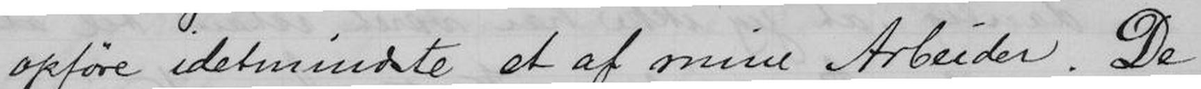opføre idemindste et af mine Arbeider. De
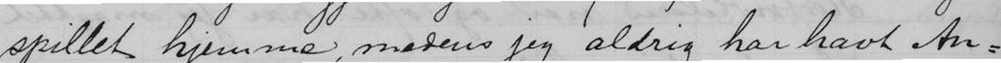spillet hjemme, medens jeg aldrig har havt An-
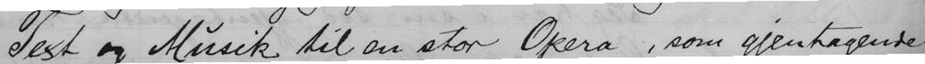Text og Musik til en stor Opera, som gjentagende
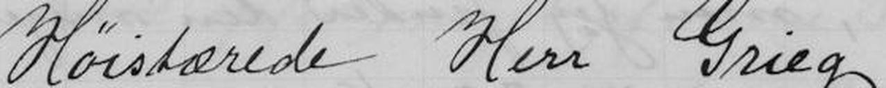Høistærede Herr Grieg!
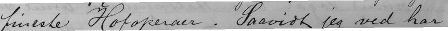fineste Hofopera. Saavidt jeg ved har
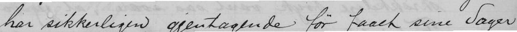har sikkerligen gjentagende før faaet sine Sager
